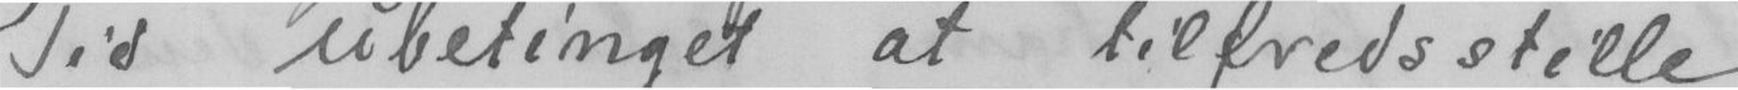Tid ubetinget at tilfredsstille
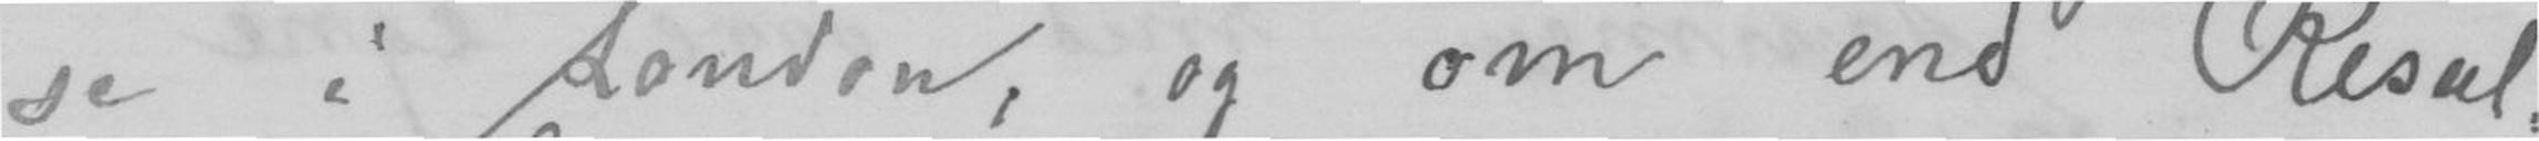se i Landen, og om end Resul-
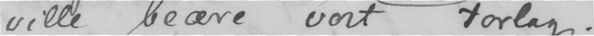ville beære vort Forlag.
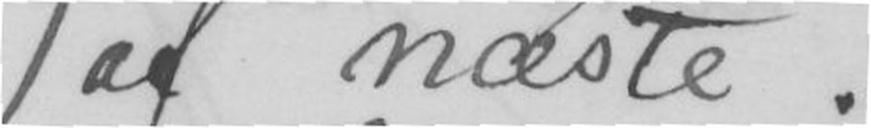af næste.
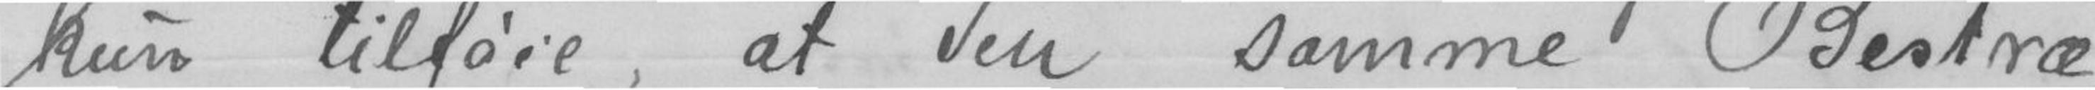kun tilføie, at den sammen Bestræ-
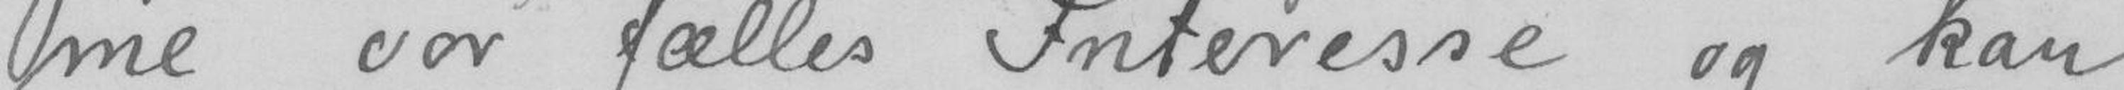me vor fælles Interesse og kan
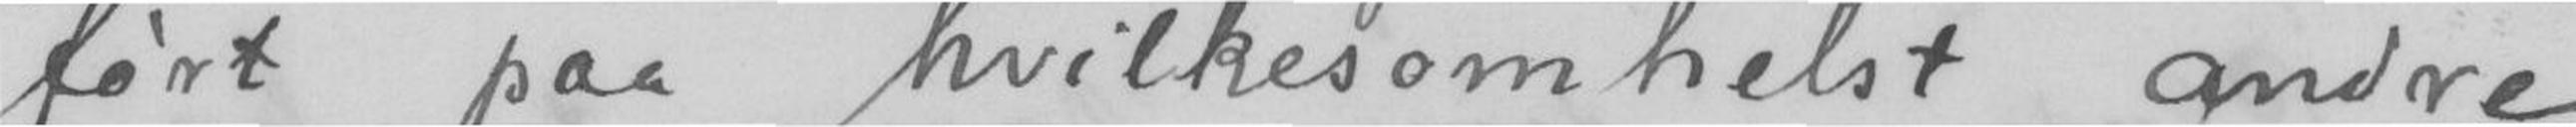ført paa hvilkesomhelst andre
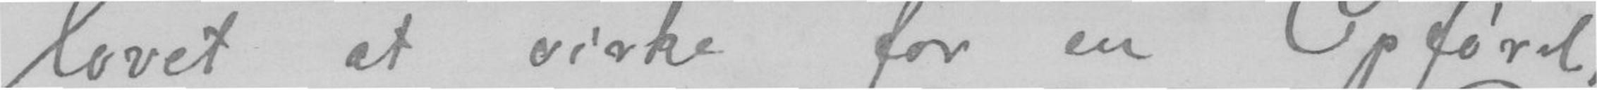lovet at virke for en Opførel-
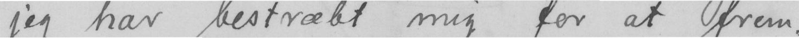jeg har bestræbt mig for at frem-
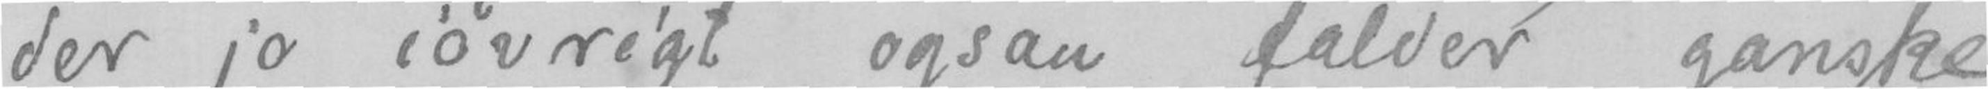der jo iøvrigt ogsaa falder ganske
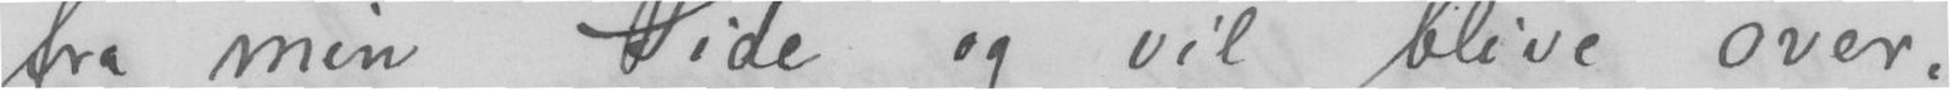fra min Side og vil blive over-
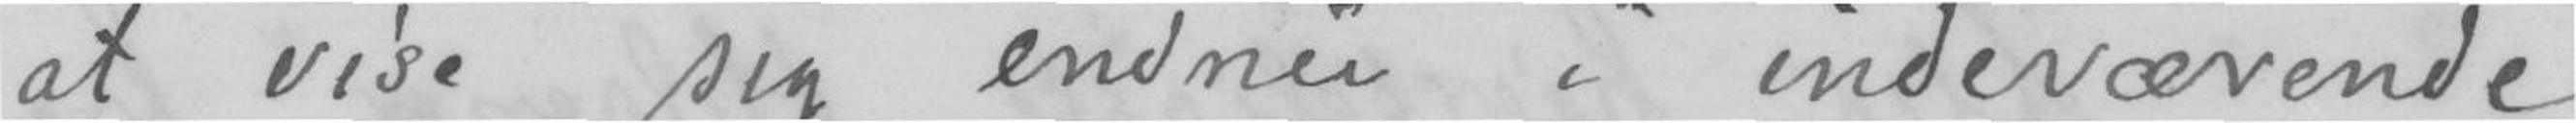at vise sig endnu i indeværende
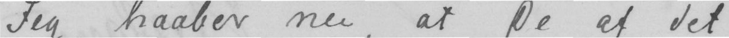Jeg haaber nu, at De af det
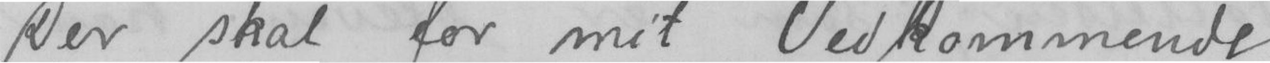Der skal for mit Vedkommende
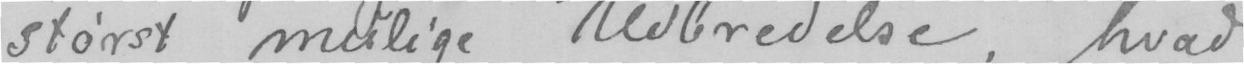størst mulige Udbredelse, hvad
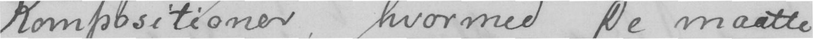Kompositioner hvormed De maatte
